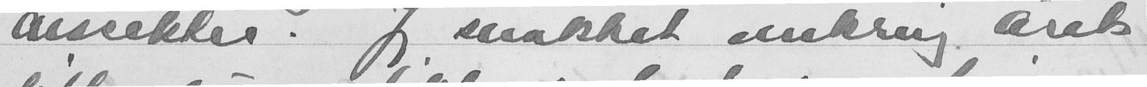ansikter. Jeg snakket omkring årets
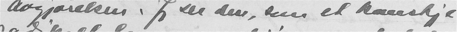avgjørelsen. Jeg ser den, som et kanskje
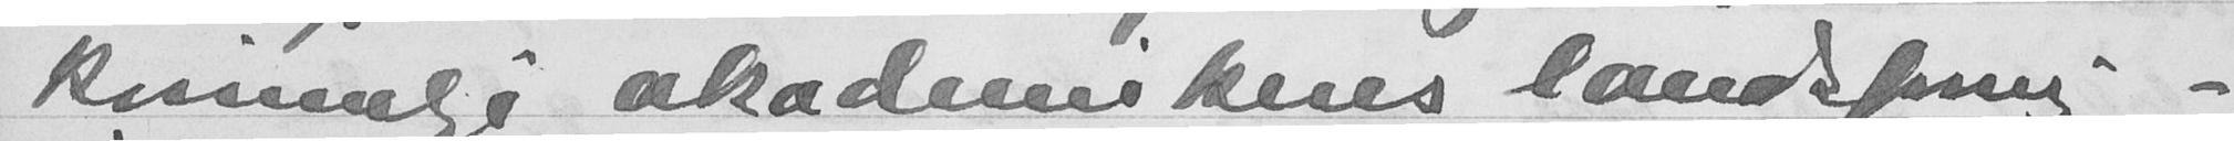Kvinnelige akademikeres landsforening -
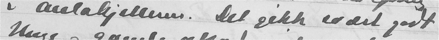i aulakjelleren. Det gikk svært godt.
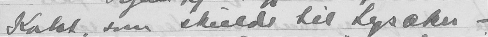Koht, som skulde til Lysaker -
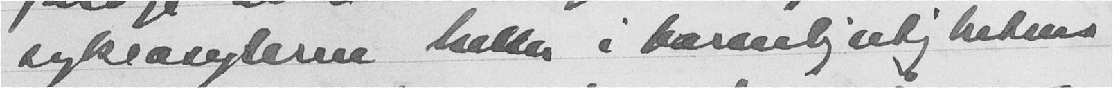sykeasylet heller i barmhjertighetens
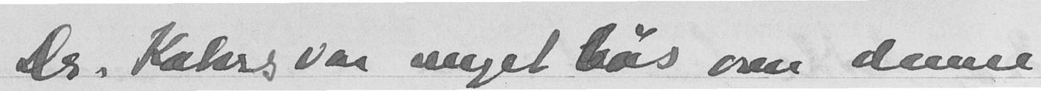Dr. Kahrs var meget høs over denne
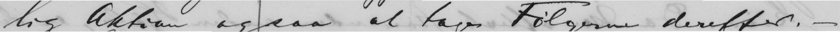lig Aktion og saa at tage Følgen derefter. -
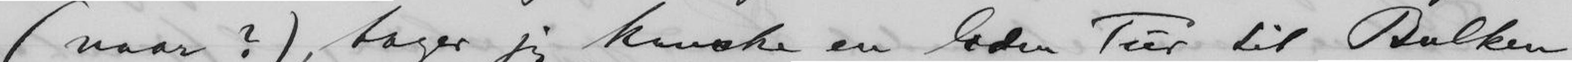(naar?), tager jeg kanske en liden Tur til Bulken
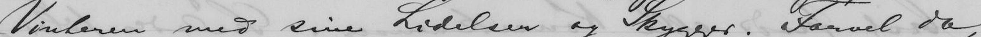Vinteren med sine Lidelser og Skygger. Farvel da,
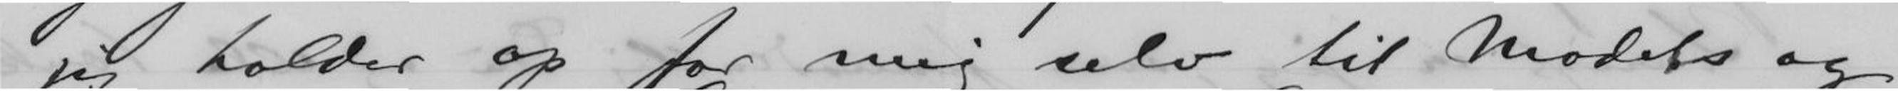jeg holder op for mig selv til Modets og
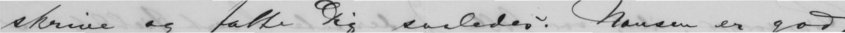skrive og fatte Dig saaledes. Nansen er god,
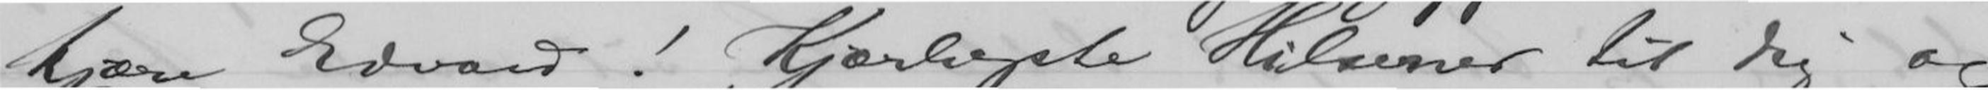kjære Edvard! Kjærligste Hilsener til Dig og
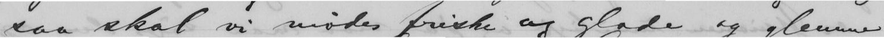saa skal vi mødes friske og glade og glemme
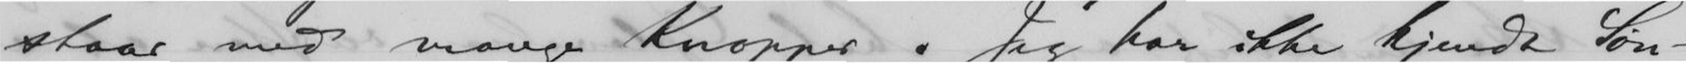staar med mange Knopper. Jeg har ikke kjendt Søn-
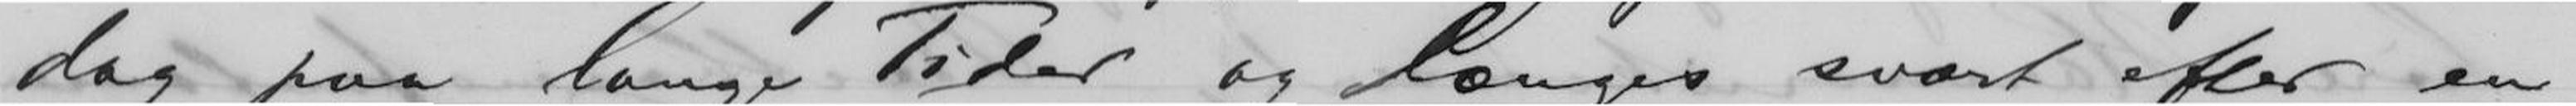dag paa lange Tider og længes svært efter en
